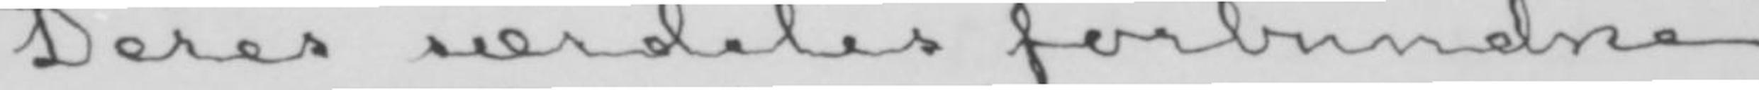Deres særdeles forbundne
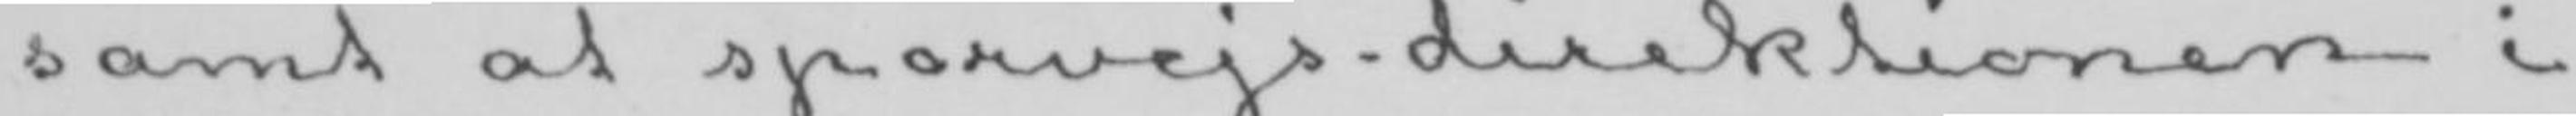samt at sporvejs-direktionen i
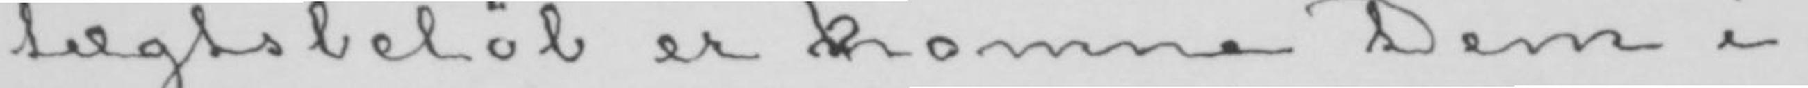tægtsbeløb er komne Dem i-
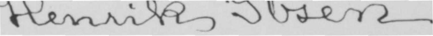Henrik Ibsen.
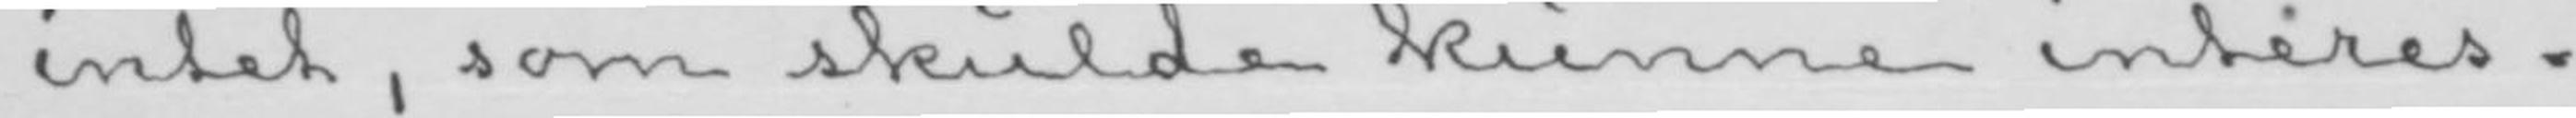intet, som skulde kunne interes-
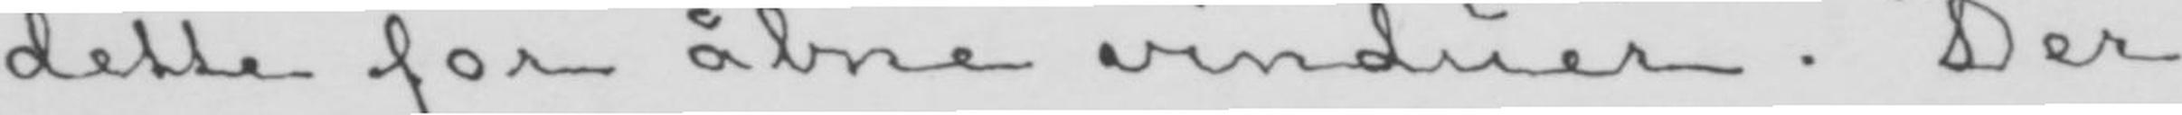dette for åbne vinduer. Der
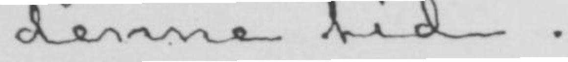denne tid.
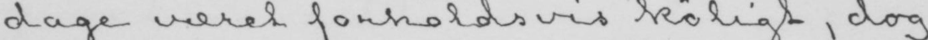dage været forholdsvis køligt, dog
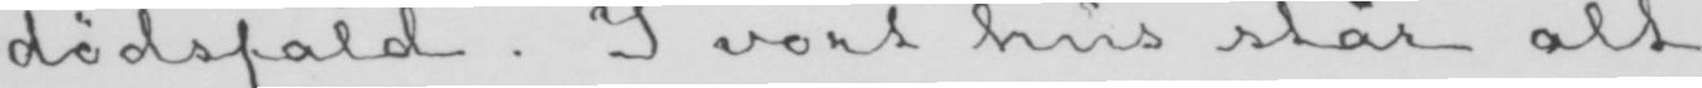dødsfald. I vort hus står alt
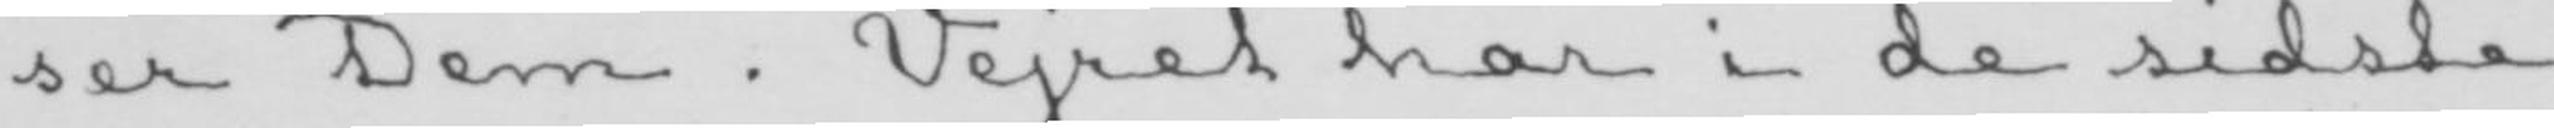ser Dem. Vejret har i de sidste
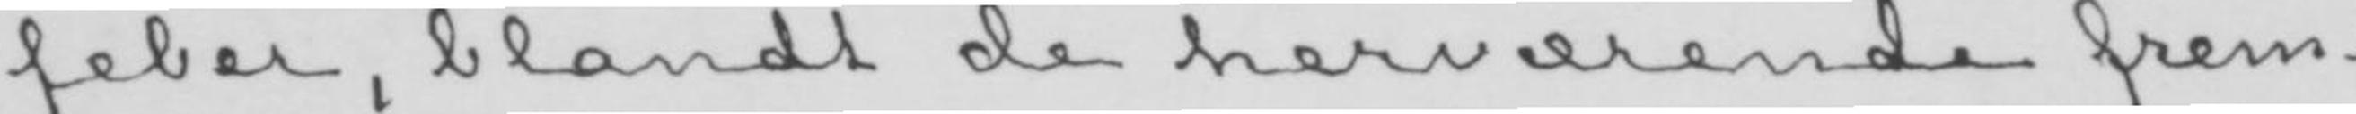feber, blandt de herværende frem-
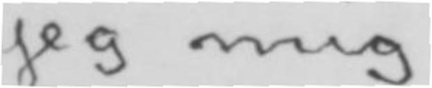jeg mig
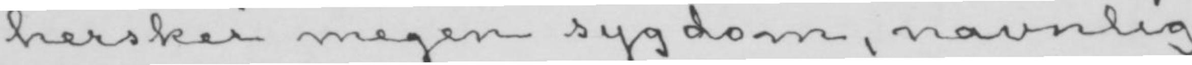hersker megen sygdom, navnlig
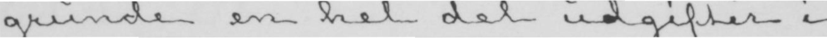grunde en hel del udgifter i
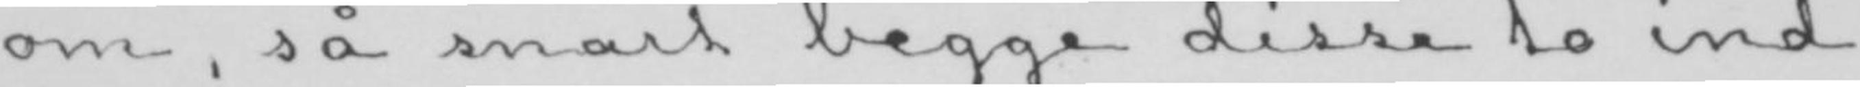om, så snart begge disse to ind-
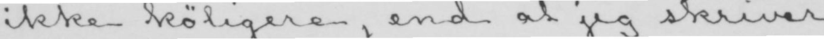ikke køligere, end at jeg skriver
